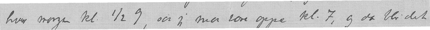hver morgen kl. ½ 9, saa jeg maa være oppe kl. 7, og da blir det
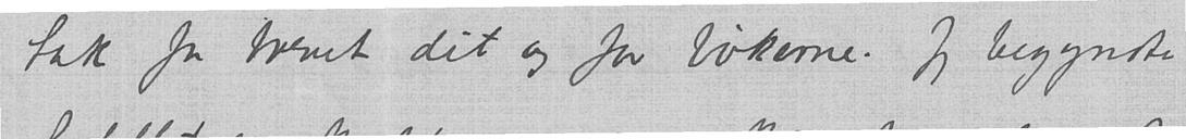tak for brevet dit og for bøkerne. Jeg begyndte
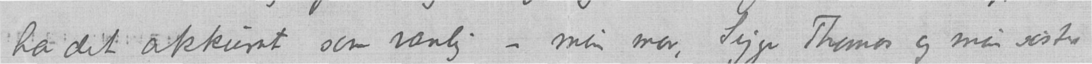ha det akkurat som vanlig – min mor, Sigge Thomas og min søster
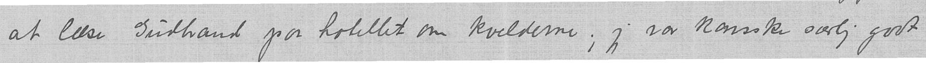at læse Gudbrand paa hotellet om kvelderne; jeg var kanske særlig godt
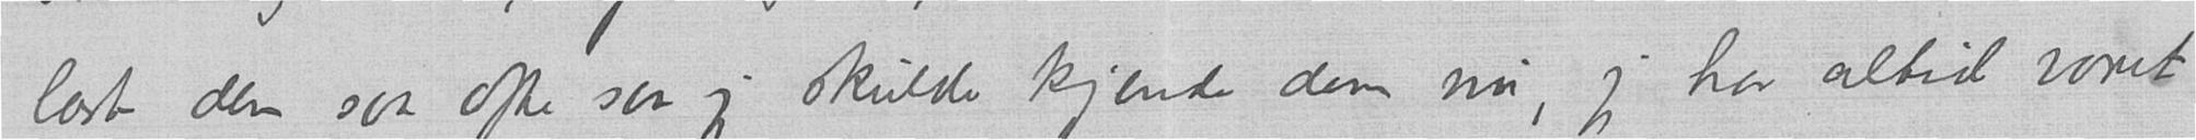læst dem saa ofte saa jeg skulde kjende dem nu, jeg har altid været
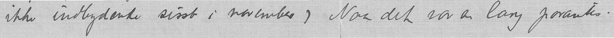ikke indbydende sisst i november) Naa det var en lang parentes.
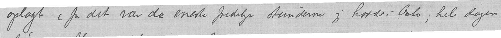oplagt (for det var de eneste fredelige stunderne jeg hadde i Oslo; hele dagen
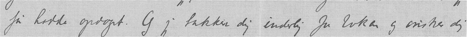før hadde opdaget. Og jeg takker dig inderlig for boken og ønsker dig
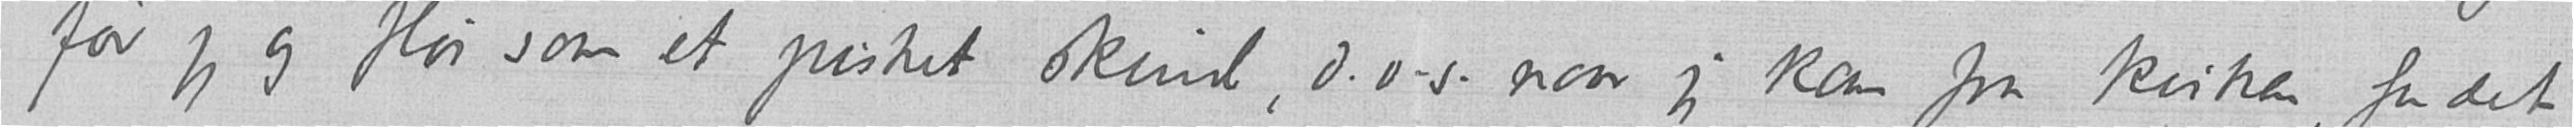for jeg og fløi som et pisket skind, d.v.s. naar jeg kom fra kirken, for det
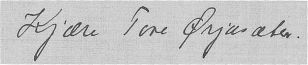Kjære Tore Ørjasæter.
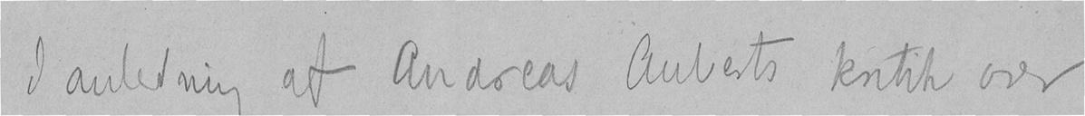I anledning af Andreas Auberts kritik over
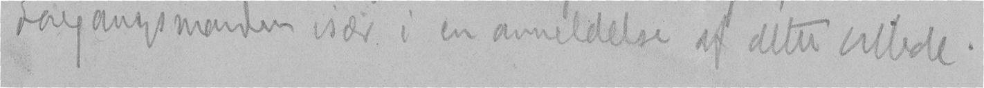Foregangsmanden især i en anmeldelse af dette billede.
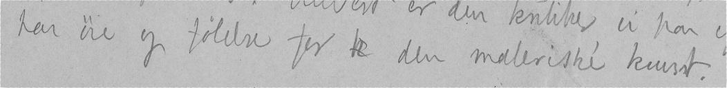har øie og følelse for k den maleriske kunst.
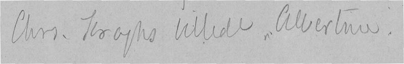Chrs. Kroghs billede "Albertine".
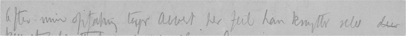Efter min opfatning tager Aubert her feil han knytter selv den
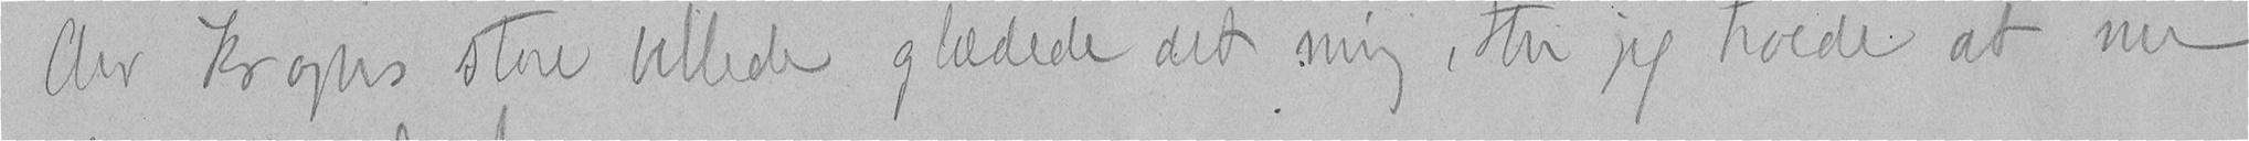Chr Kroghs store billede glædede det mig, thi jeg troede at nu
